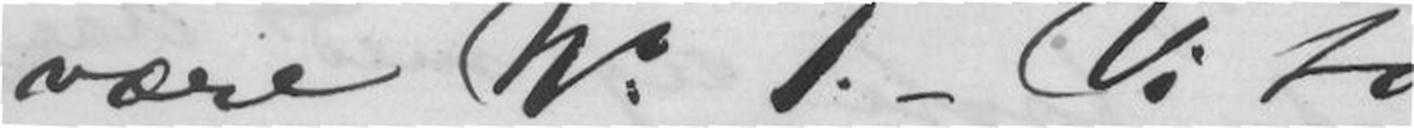være N. 1. - Vi to
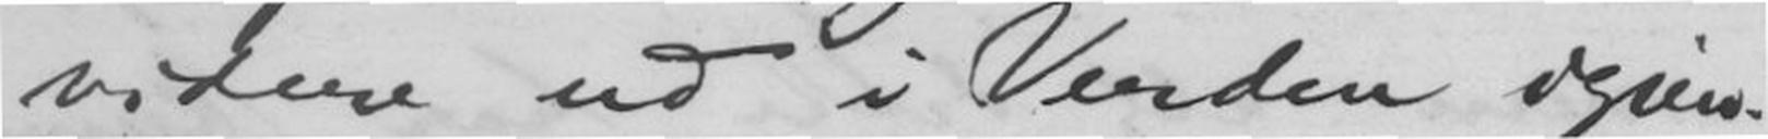videre ud i Verden igjen.
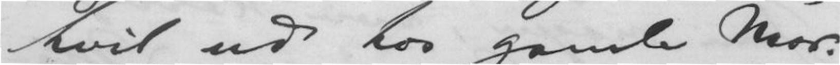hvil ud hos gamle Mor.
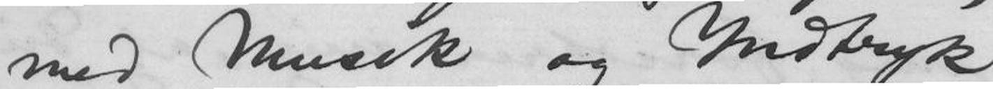med Musik og Indtryk
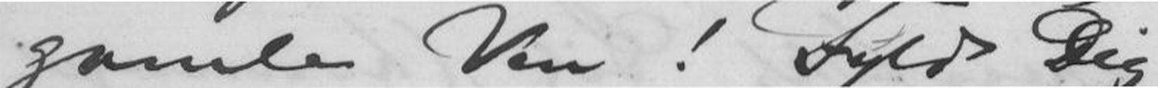gamle Ven! Fyld Dig
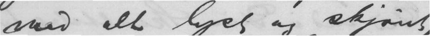med alt lyst og skjønt,
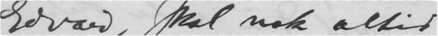Edvard, skal nok altid
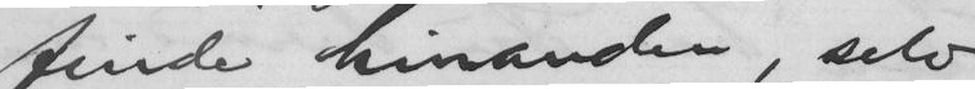finde hinanden, selv
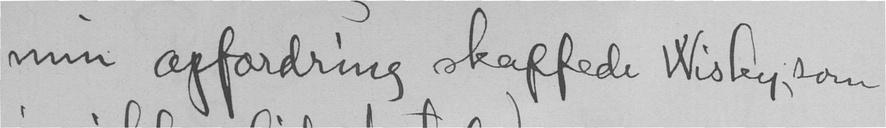min opfordring skaffede Wisky, som
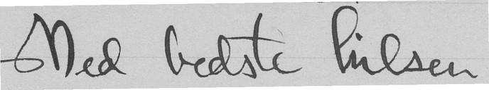Med bedste hilsen
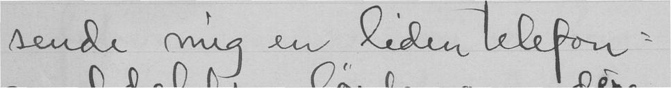sende mig en liden telefon-
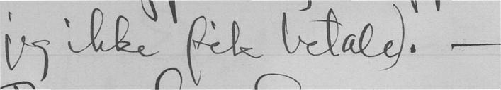jeg ikke fik betale). -
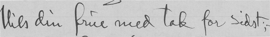tils den frue med tak for sidst; -
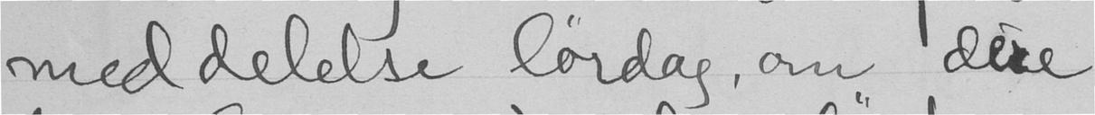meddelelse lørdag, om dere
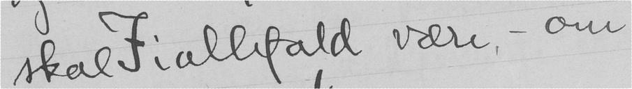skal I iallefald være, - om
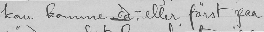kan komme da, - eller først paa
